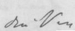Din Ven
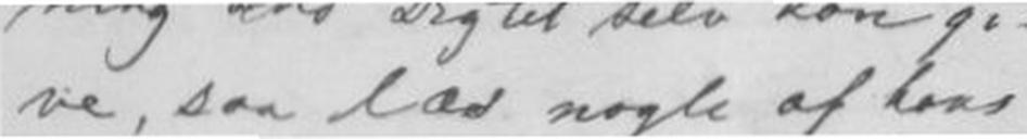ve, saa lad nogle af hans
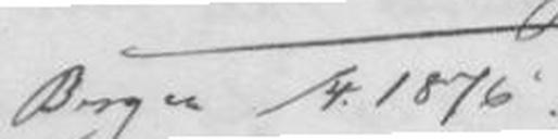Bergen /4 1876
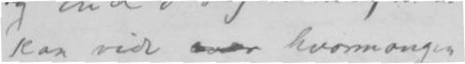kan vide hvormangen
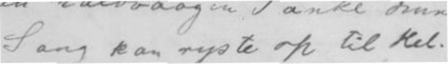Sang kan ryste op til Hel-
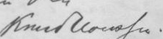Knud Claussen
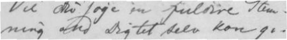ning end Digtet selv kan gi-
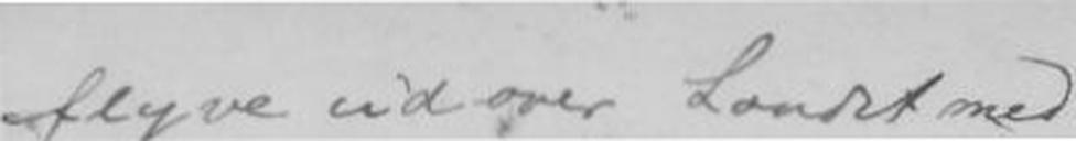flyv udover Landet med
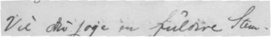Vil Du søge en fuldøve Stem-
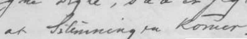at Stemningen kommer.
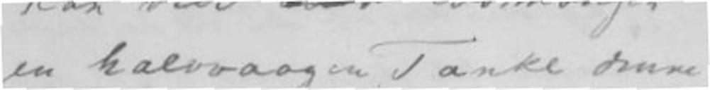en halvvaagen Tanke denne
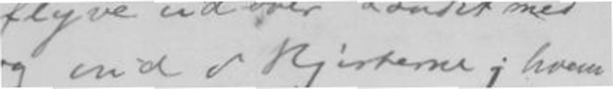og ind i Hjerterne; hvem
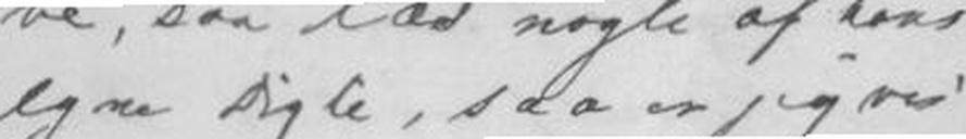egne Digte, saa er jeg vis
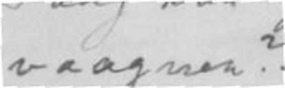vaagnen?
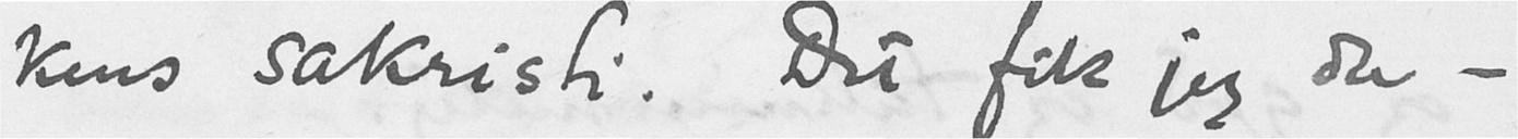kens sakristi. Det fik jeg da -
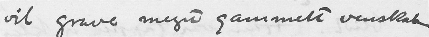vil grave meget gammelt venskab
